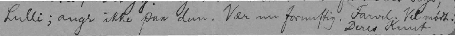Lulli; angre ikke paa dem. Vær nu fornuftig. Farvel. Vel mødt.
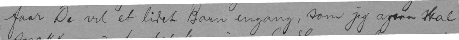faar De vel et lidet Barn engang, som jeg ogsaa skal
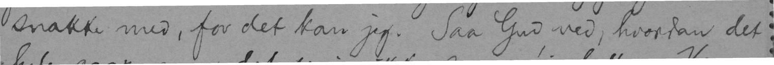snakke med, for det kan jeg. Saa Gud ved, hvordan det
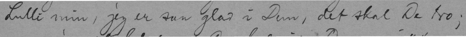Lulli min, jeg er saa glad i Dem, det skal De tro;
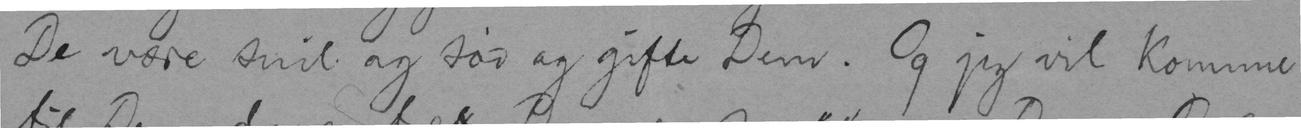De være snil og sød og gifte Dem. Og jeg vil komme
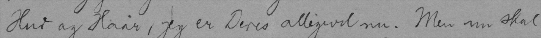Hud og Haar, jeg er Deres alligevel nu. Men nu skal
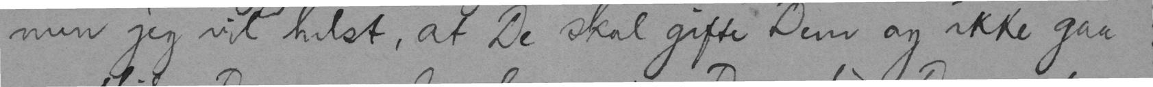men jeg vil helst, at De skal gifte Dem og ikke gaa
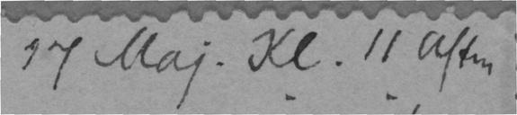17 Maj. Kl. 11 Aften
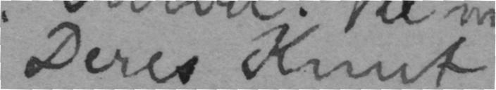Deres Knut
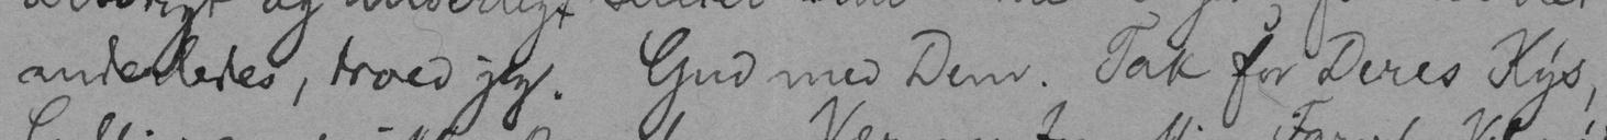anderledes, troed jeg. Gud med Dem. Tak for Deres Kys,
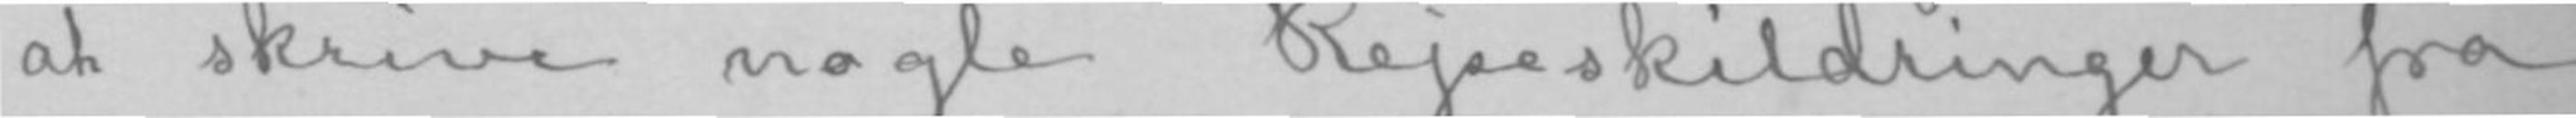at skrive nogle Rejseskildringer fra
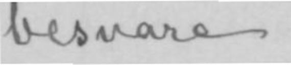besvare.
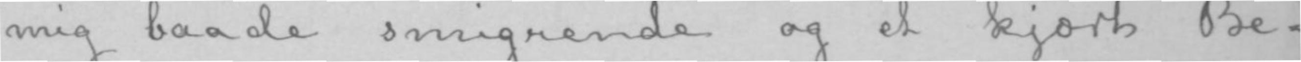mig baade smigrende og et kjært Be-
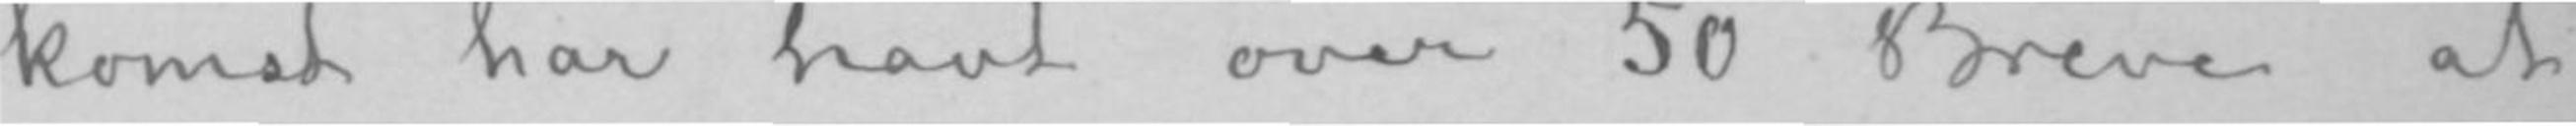komst har havt over 50 Breve at
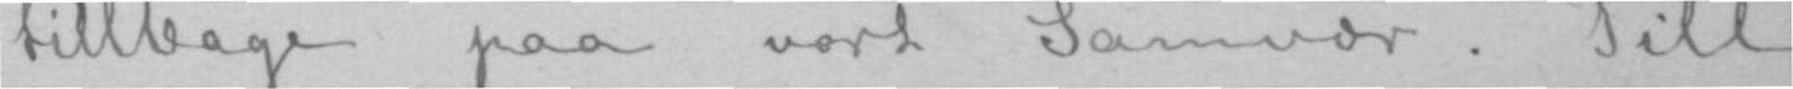tillbage paa vort Samvær. Till
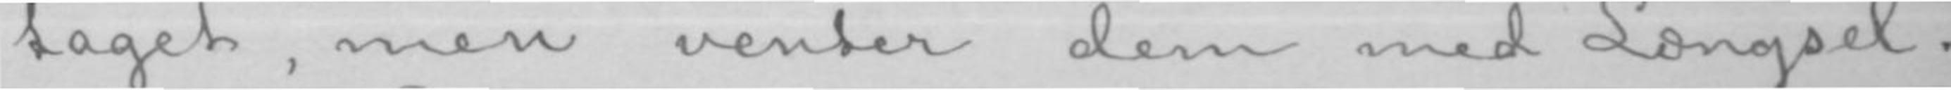taget, men venter dem med Længsel.
